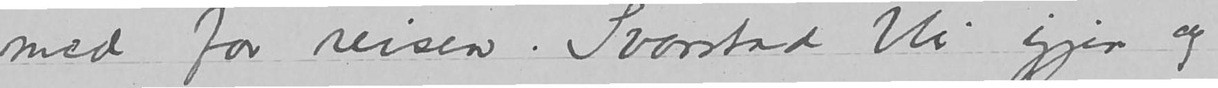med for reisen. Svarstad blir igjen og
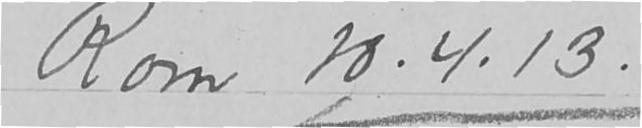Rom, 10.4.13.
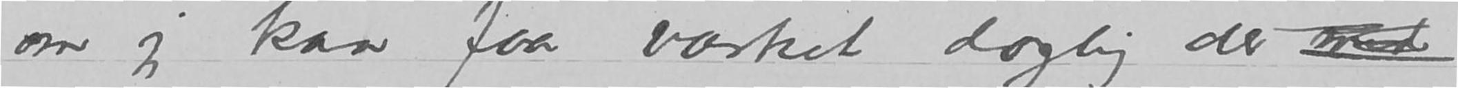om jeg kan faa vasket daglig der med
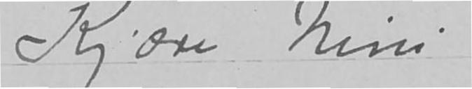Kjære Nini,
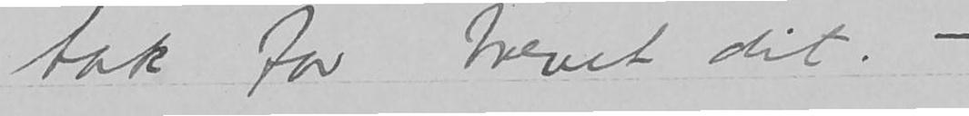tak for brevet dit. -
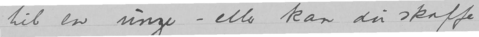til en unge – eller kan du skaffe
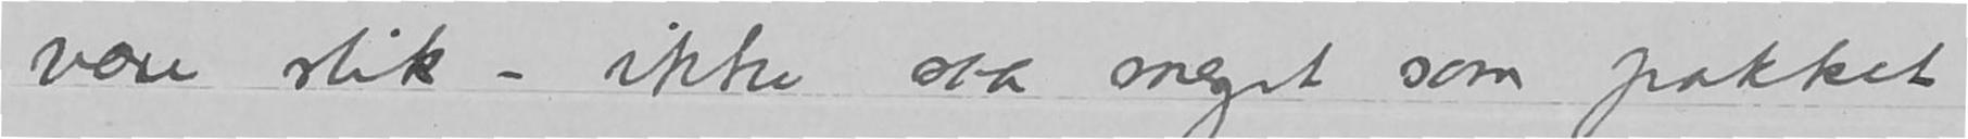være slik – ikke saa meget som pakket
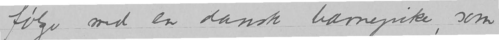følge med en dansk barnepike, som
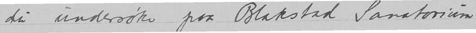du undersøke paa Blakstad Sanatorium,
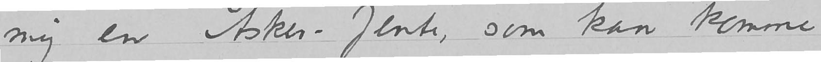mig en Asker-Jente, som kan komme
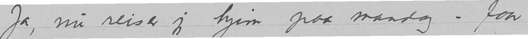Ja, nu reiser jeg hjem paa mandag - faar
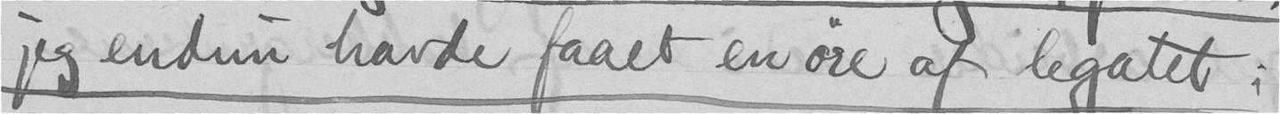jeg endnu havde faaet en øre af legatet;
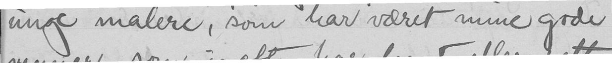unge malere, som har været mine gode
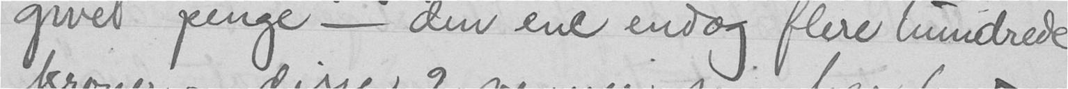givet penge - den ene endog flere hundreds
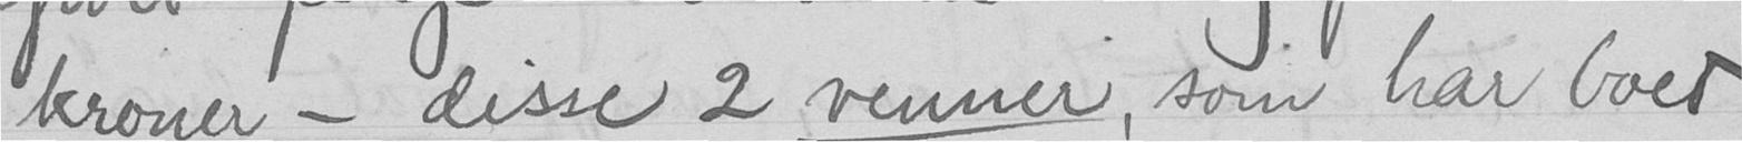kroner - disse 2 venner, som har boet
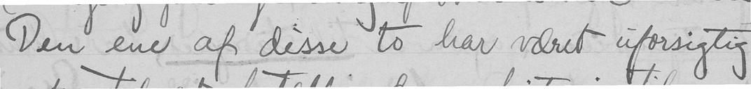Den ene af disse to har været uforsigtig
